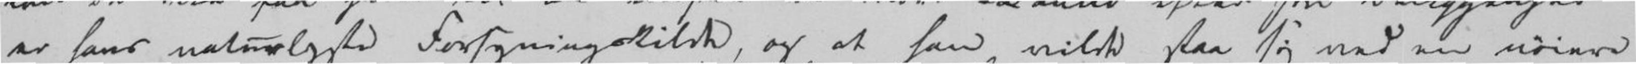er hans narturligste Forsyningskilde, og at han vilde staa sig ved en nøiere
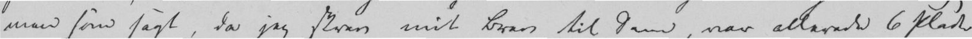men som sagt, da jeg skrev mit Brev til Dem, var allerede 6 Plade
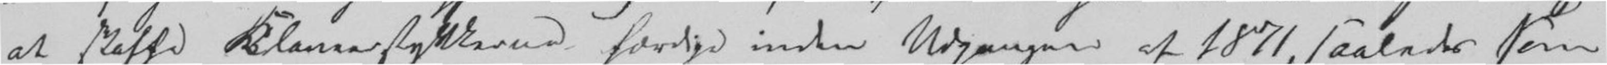at skaffe Klaveerstykkerne færdige inden Udgangen af 1871, saaledes som
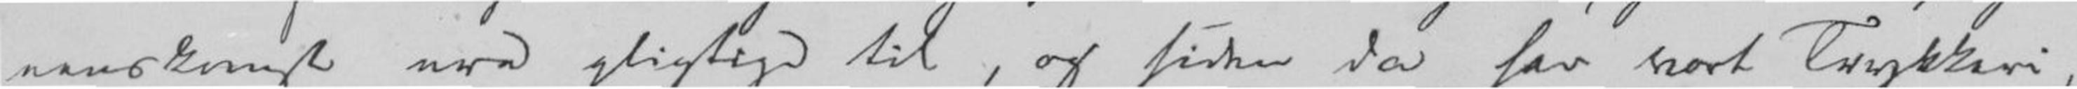renskomst ere pligtige til, og siden da har vort Trykkeri, -
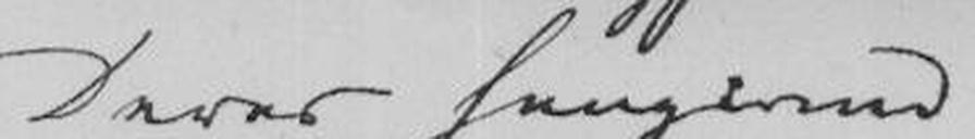Deres hengivne
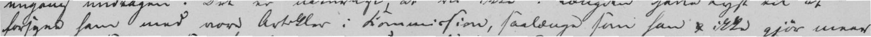forsyne ham med vore Artikler i Commission, saalænge som han ikke gjør meer
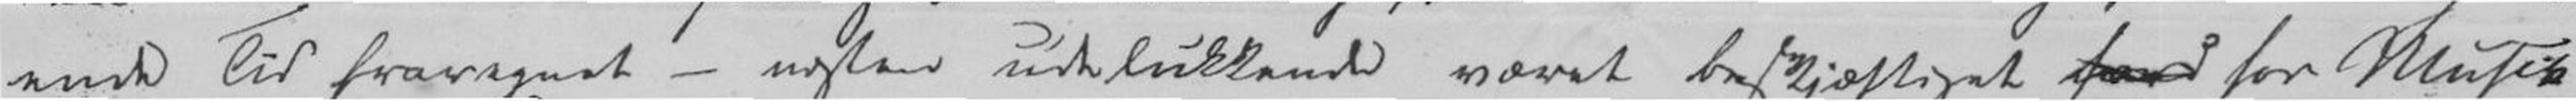ende Tid fraregnet - næsten udelukkende været beskjæftiget for Musik-
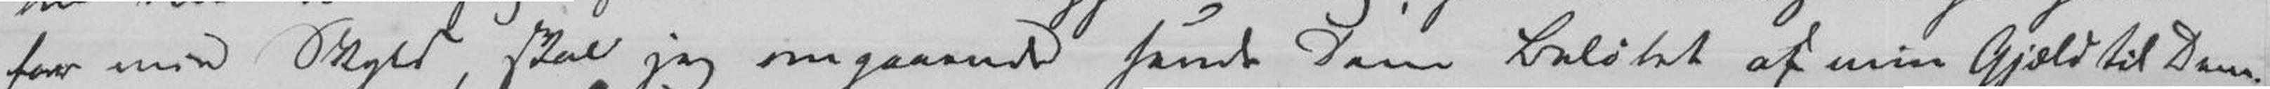for min Skyld, skal jeg omgaaende sende Dem Beløbet af min Gjæld til Dem.
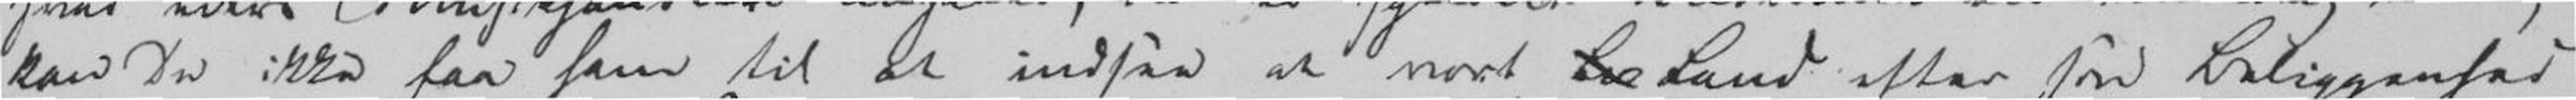kan Du ikke faa ham til at indsee at vort Land efter sin Beliggenhed
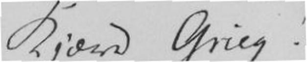Kjære Grieg!
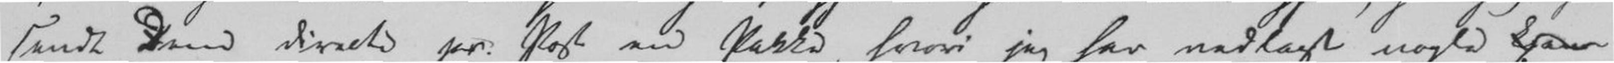sendt Dem directe pr. Post en Pakke, hvori jeg har nedlagt nogle Exem
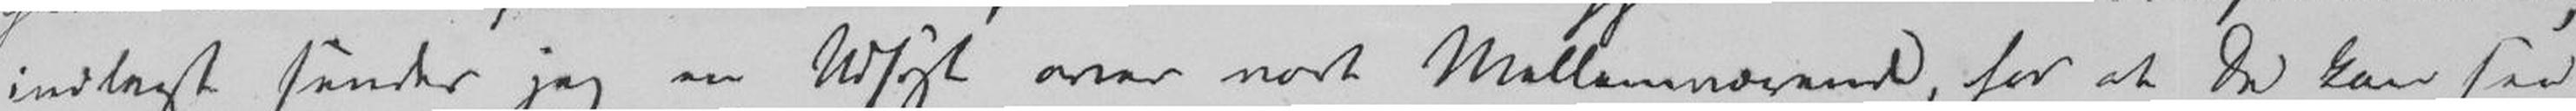indlagt sender jeg en Udsigt over vort Mellemværende, for at De kan see
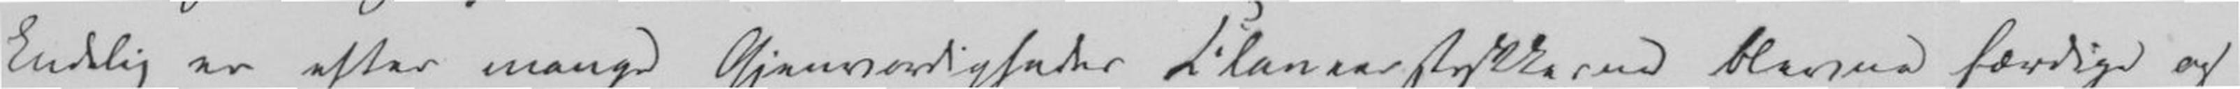Endelig er efter mange Gjenvordigheder Claveerstykkerne blevne færdige og
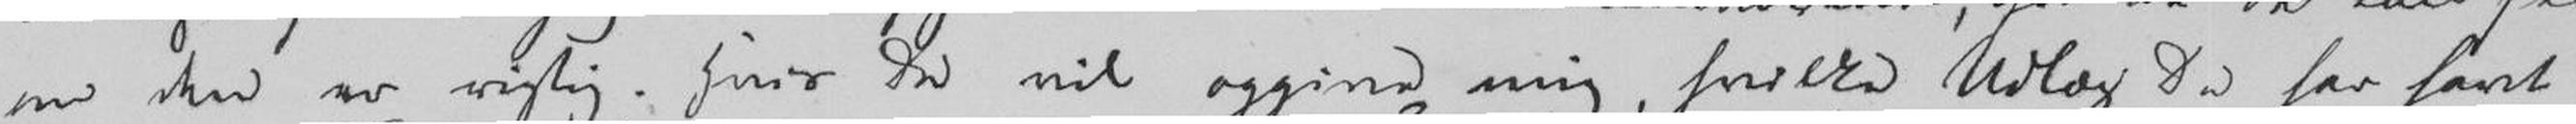om den er rigtig. Hvor De vil Opgive mig, hvilke Udlæg De har havt
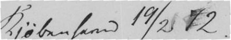Kjøbenhavn 19/2 72.
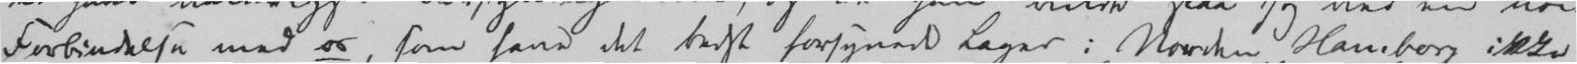Forbindelse med os, som have det bedst forsynede Lager: Norden, Hamborg ikke
